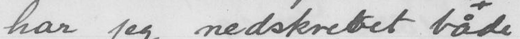har jeg nedskrevet både
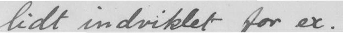lidt indviklet for ex.
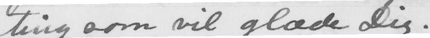ting som vil glæde Dig.
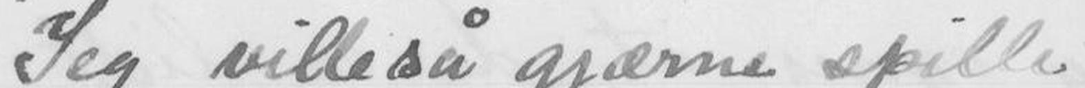Jeg ville så gjærne spille
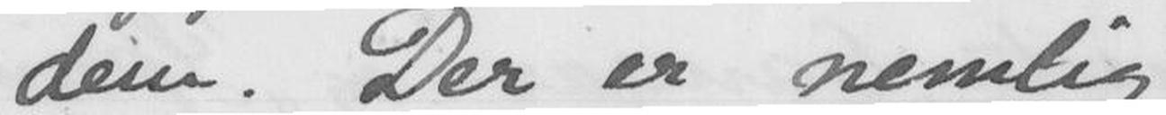dem. Der er nemlig
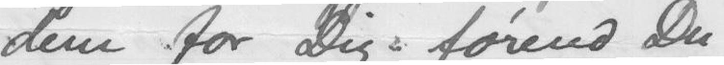dem for Dig førend Du
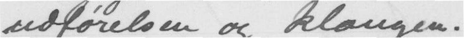udførelsen og klangen.
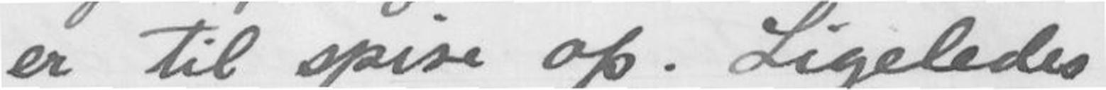er til spise op. Ligeledes
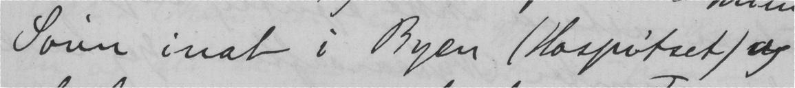Søvn i nat i Byen (Hospitset) og
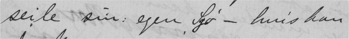seile sin egen Sjø - hvis han
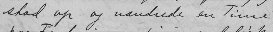stod op og vandrede en Time
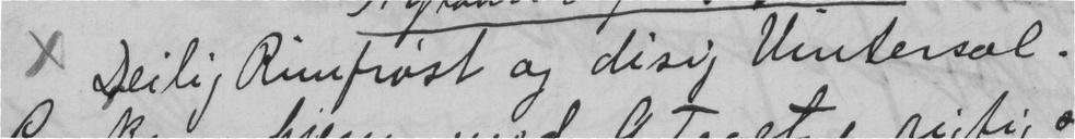x Deilig Rimfrost og disig Vintersol.
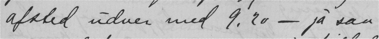afsted udver med 9. 20 - ja saa
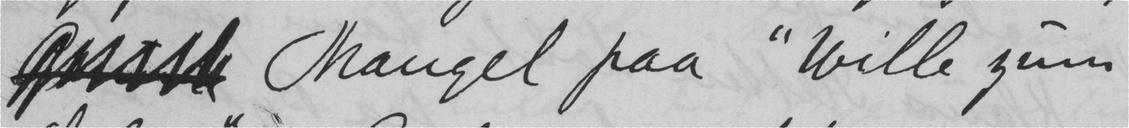mangel paa "Wille zum
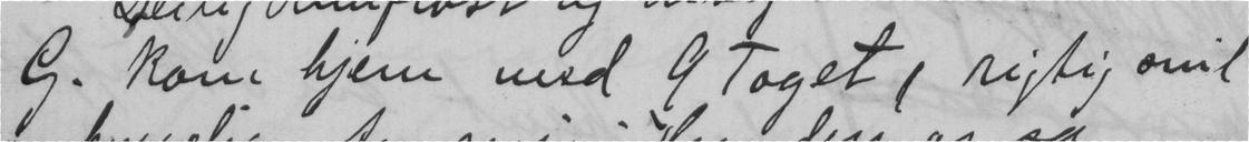G. kom hjem med 9 Toget, rigtig snil
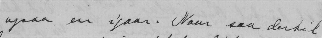ogsaa en igaar. Naar saa dertil
